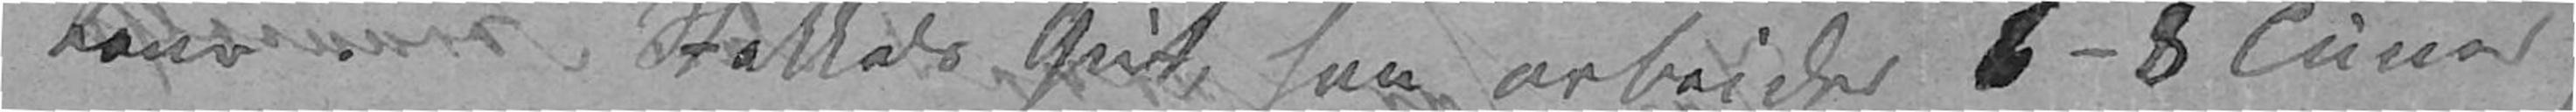tour? Stakkels Gut, han arbeider 6–8 Timer
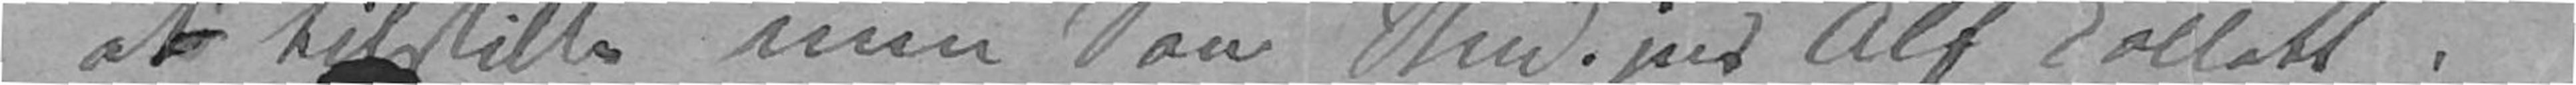at tilstille min Søn Stud. jus Alf Collett,
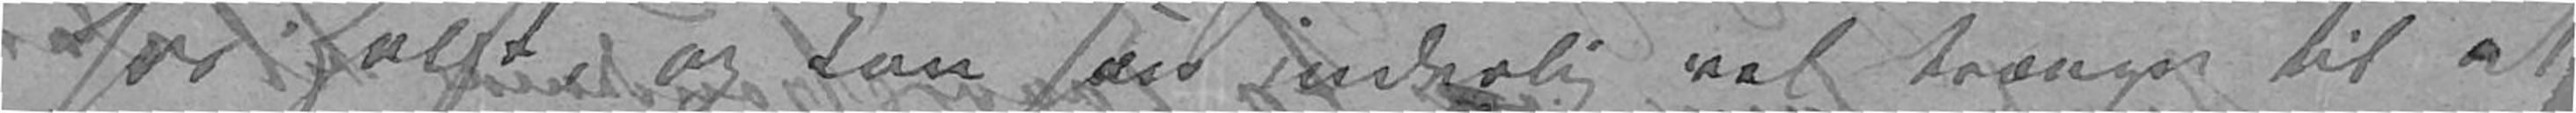hos Holst, og kan saa inderlig vel trænge til at
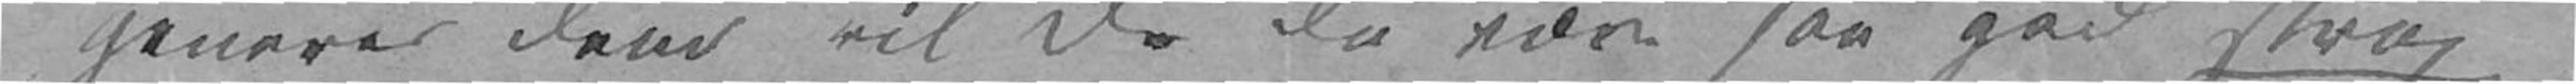generer Dem vil De da være saa god strax
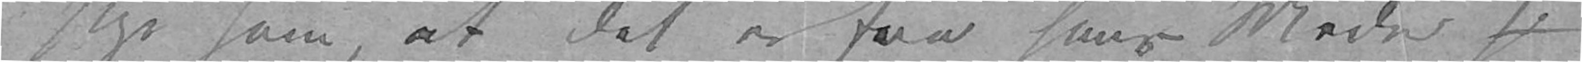sige ham, at det er fra hans Moder
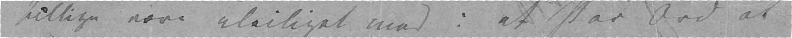tillige være uleiliget med i et Par Ord at
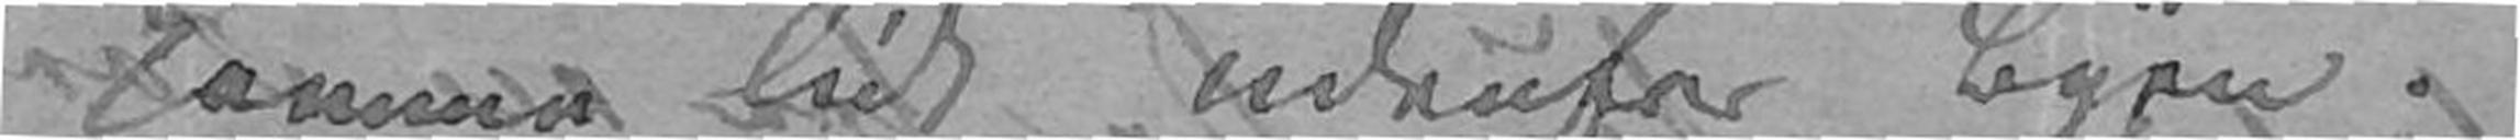komme lidt udenfor Byen.
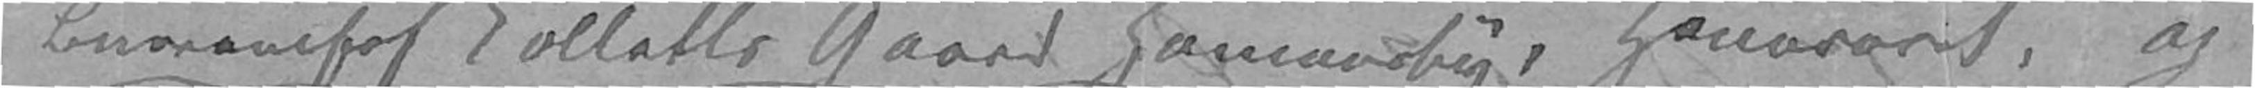Bureauchef Colletts Gaard Homansby, Honoraret, og
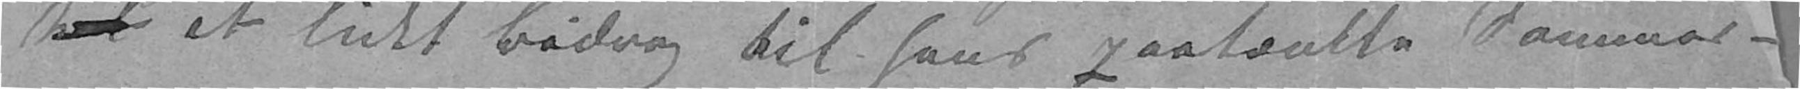<gap /><gap /></del> et lidet Bidrag til hans paatænkte Sommer¬
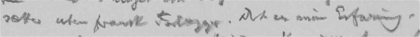sætter uten fransk Forlægger. Det er min Erfaring.
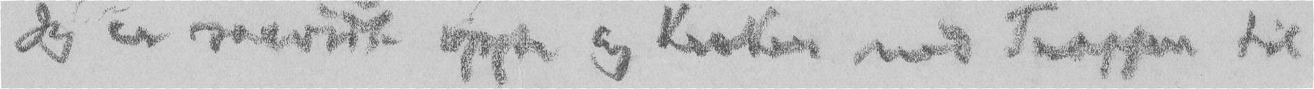Jeg er saavidt oppe og kræker mod Trappen til
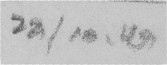22/10. 49.
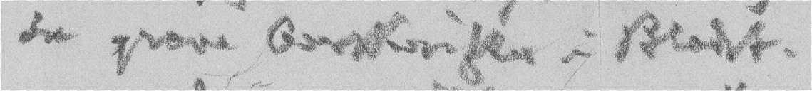de grove Overskrifter i Bladet.
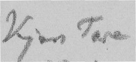Kjære Tore
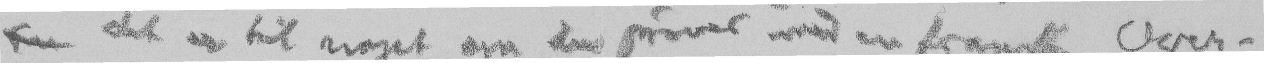han det er til noget om du prøver med en fransk Over-
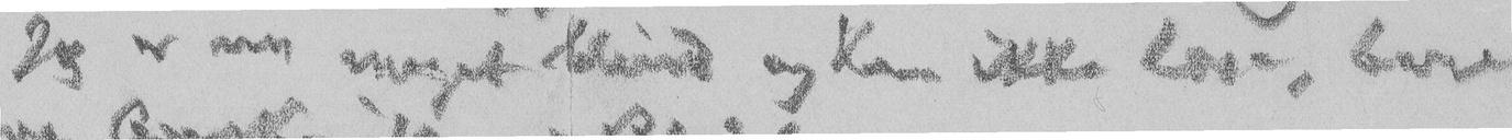Jeg er nu meget blind og kan ikke læse, bare
